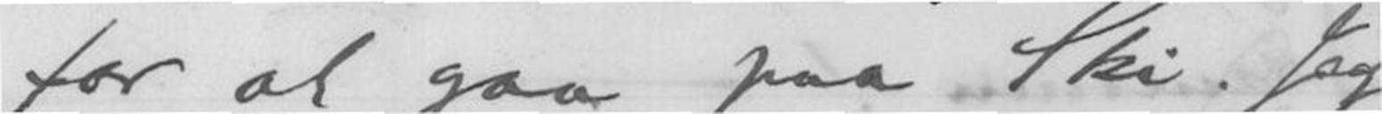for at gaa paa Ski. Jeg
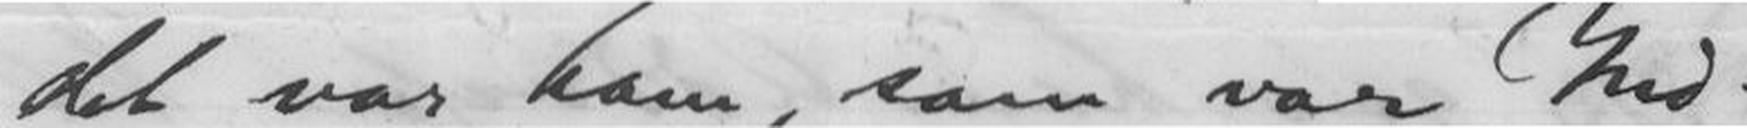det var ham, som var Ind-
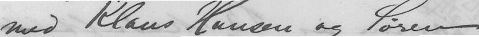med Klaus Hansen og Søren
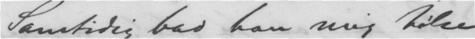Samtidig bad han mig hilse
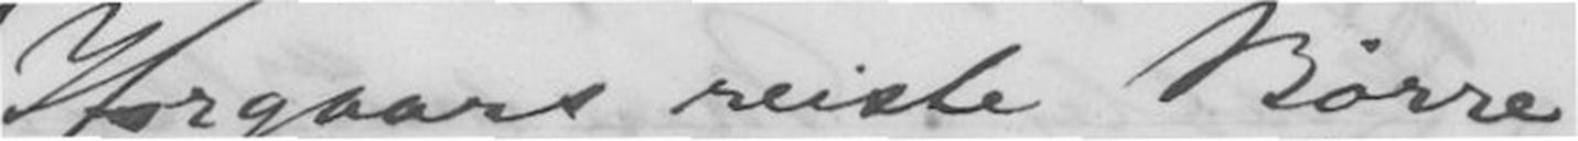Iforgaars reiste Børre
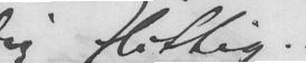dig flittig. -
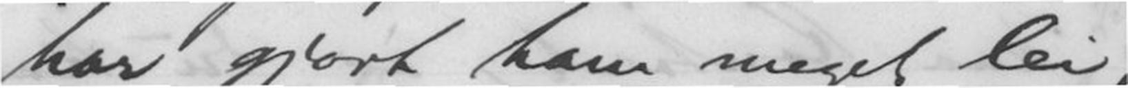har gjort ham meget lei,
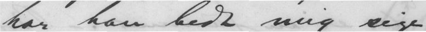har han bedt mig sige
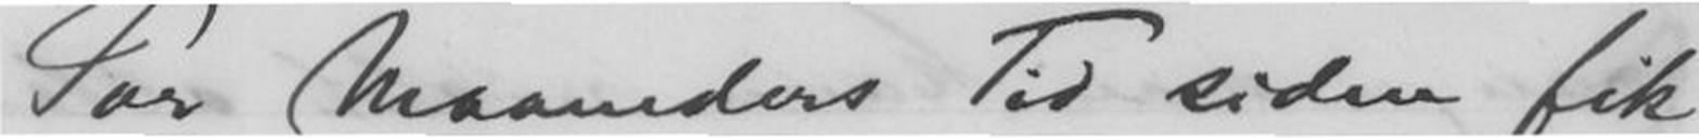Par Maaneders Tid siden fik
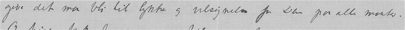give det maa bli til lykke og velsignelse for Dem paa alle maater.
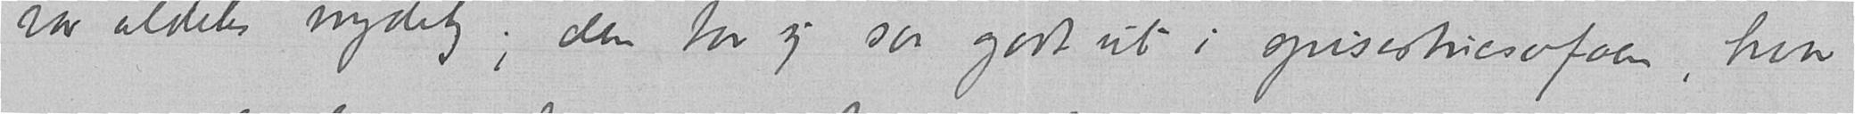var aldeles nydelig; den tar sig saa godt ut i spisestuesofaen, hvor
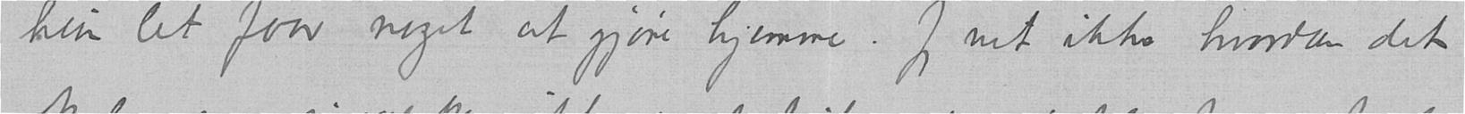hun let faar noget at gjøre hjemme. Jeg vet ikke hvordan det
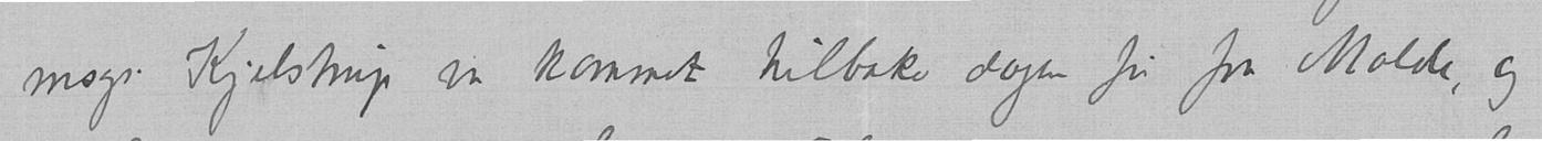msgr. Kjelstrup var kommet tilbake dagen før fra Molde, og
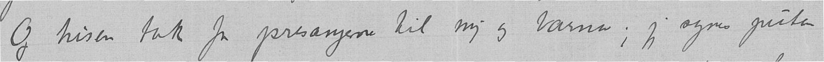Og tusen tak for presangerne til mig og barna; jeg synes puten
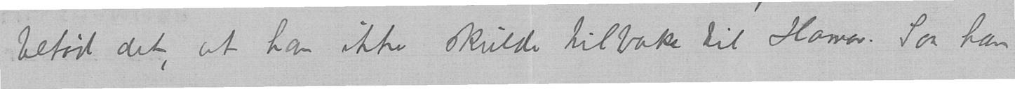betød det, at han ikke skulde tilbake til Hamar. Saa han
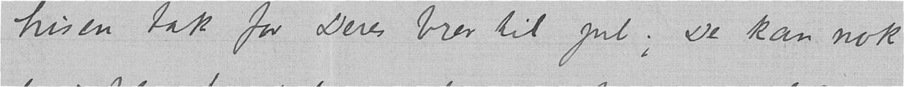tusen tak for Deres brev til jul; De kan nok
